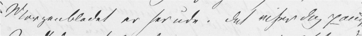Morgenbladet er her ude. Det viser dog pent
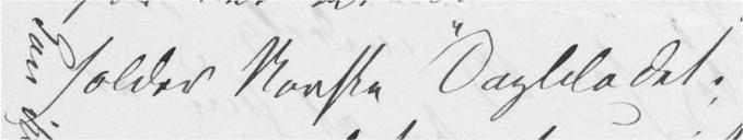holdes Norske "Dagbladet."
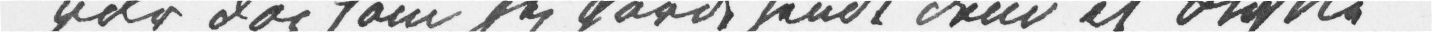var dog som jeg havde sendt dem et Stykke
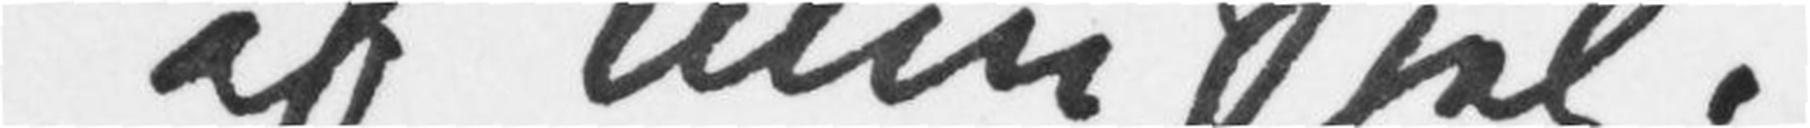af min Sjel.
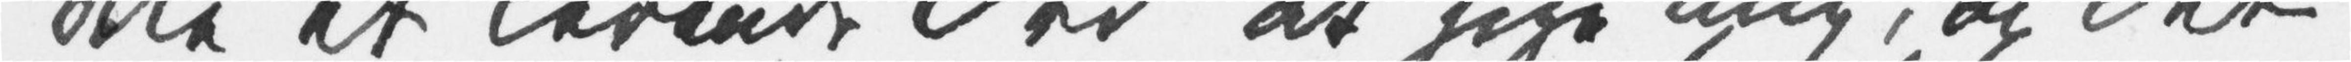ikke et levende Ord at sige mig, og det
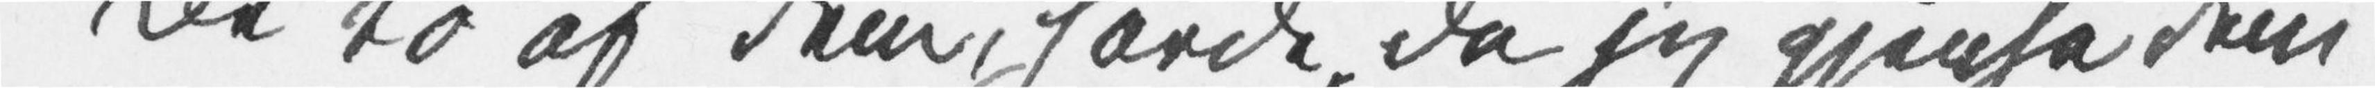De to af dem, havde, da jeg gjenså dem
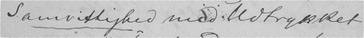Samvittighed med Udtrykket
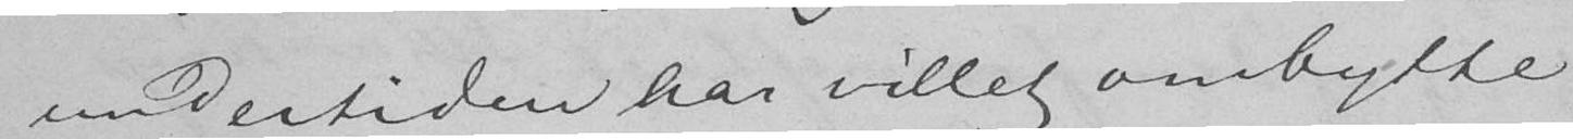undertiden har villet ombytte
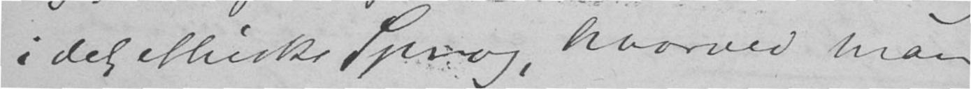i det ethiske Sprog, hvorved man
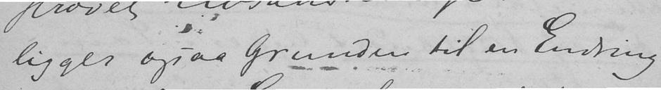ligger ogsaa Grunden til en Endring
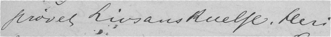prøvet Livsanskuelse. Heri
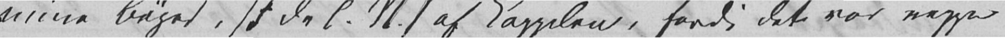mine Bøger, (I de l. N.) af Cappelen, fordi det var neppe
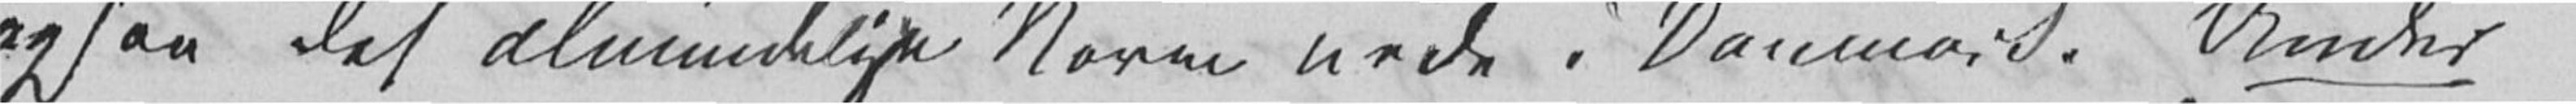ogsaa det almindelige Norm nede i Danmark. Under
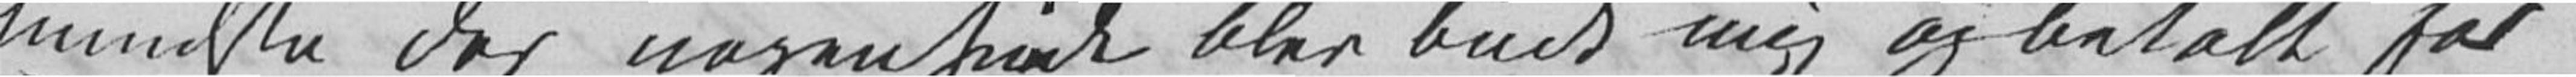mindste der nogensinde blev budt mig og betalt for
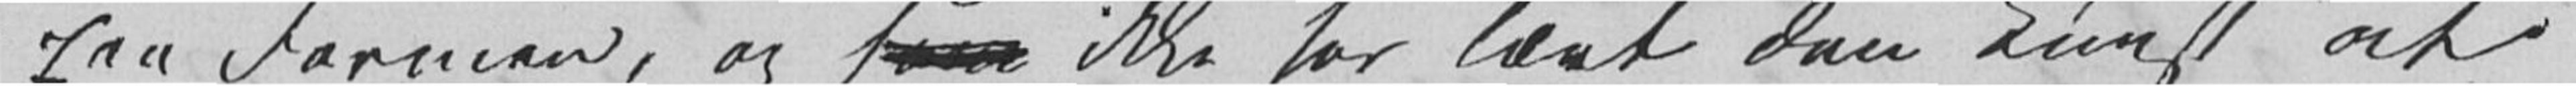paa Formen, og som ikke har lært den Kunst at
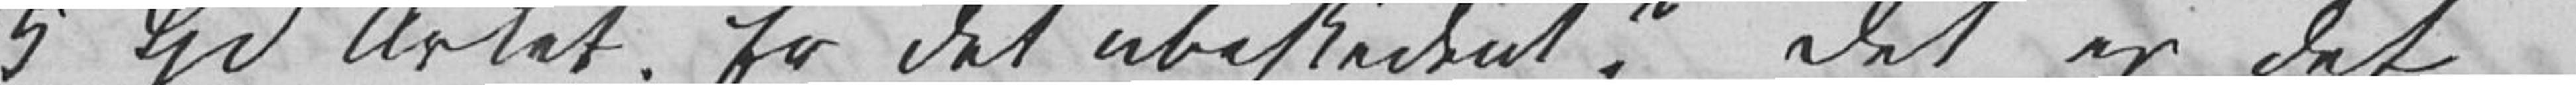15 Spd Arket. Er det ubeskedent? Det er det
